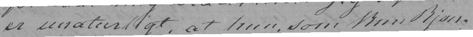er unaturligt, at hun som kun kjen-
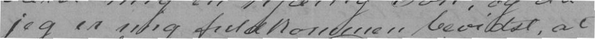jeg er mig fuldkommen bevidst, at
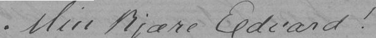Min kjære Edvard!
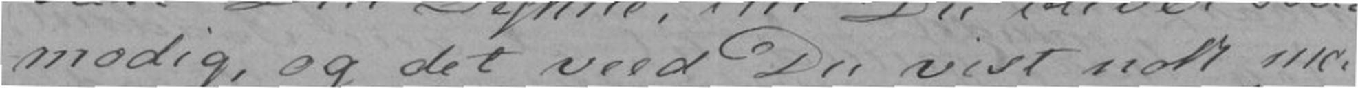modig, og det veed Du vist nok me-
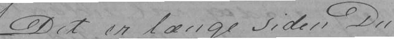Det er længe siden Du
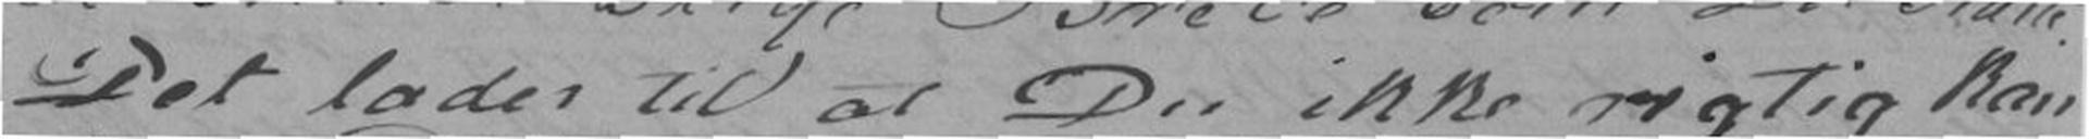Det lader til at Du ikke rigtig kan
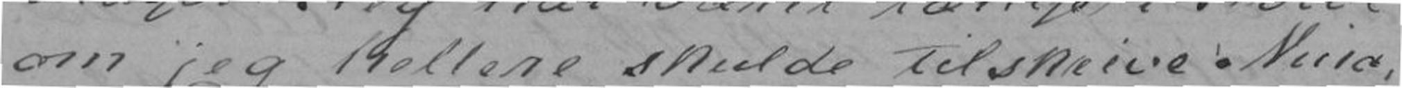om jeg hellere skulde tilskrive Nina,
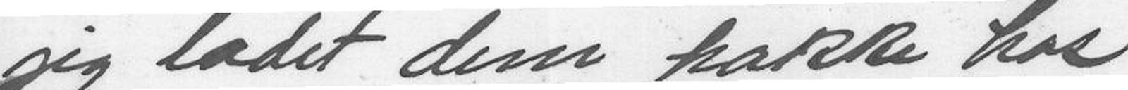jeg ladet dem pakke hos
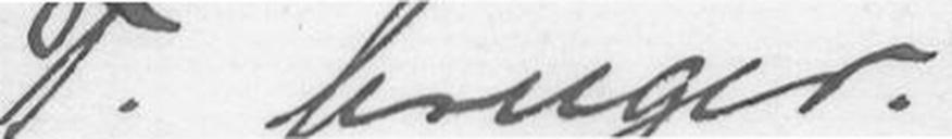T. bruger.
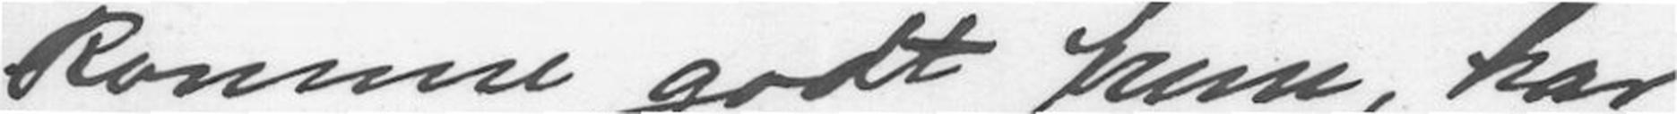komme godt frem, har
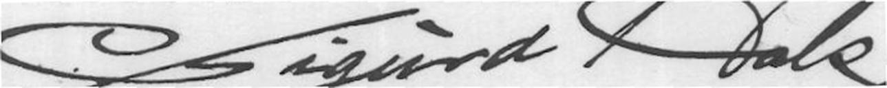Sigurd Hals
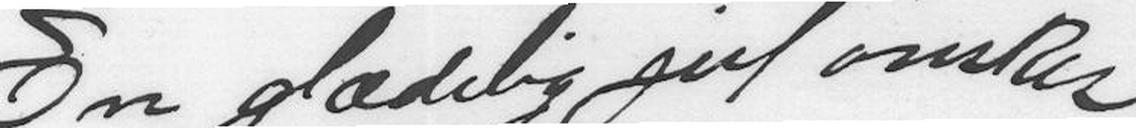En glædelig jul ønskes
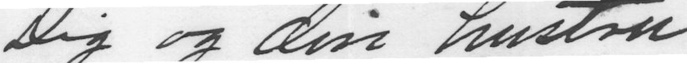Dig og din hustru
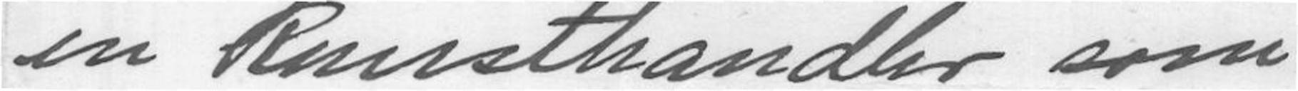en kunsthandler som
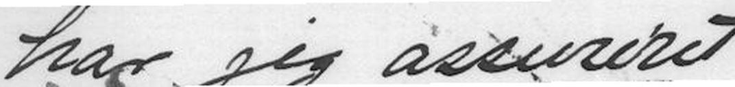har jeg assureret
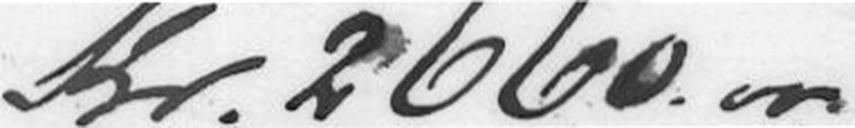Kr. 2660.oo
